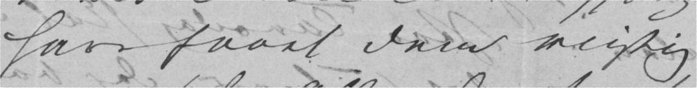have faaet dem rigtig
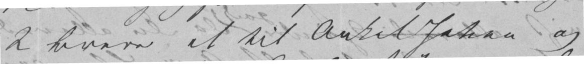2 Breve et til Onkel Johan og
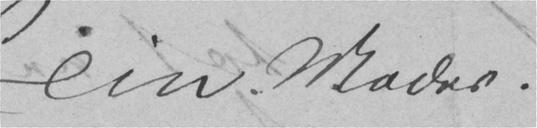Din Moder
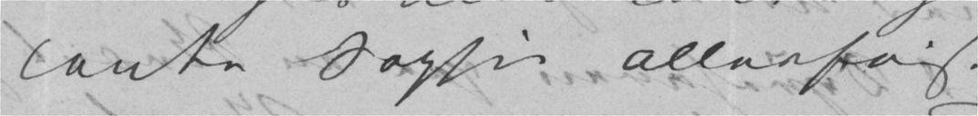Tante Sophie allerførst.
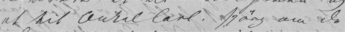et til Onkel Carl, spørg om de
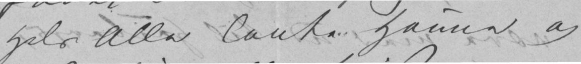Hils Alle Tante Hanne og
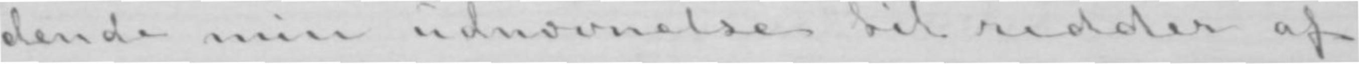dende min udnævnelse til ridder af
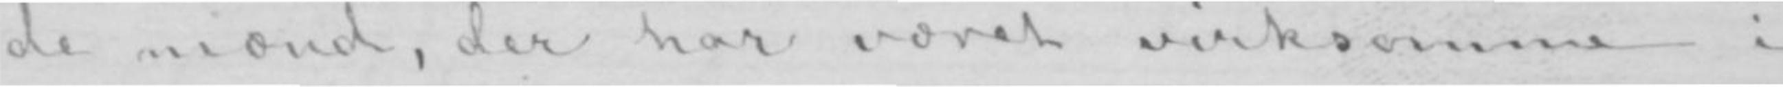de mænd, der har været virksomme i
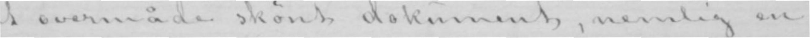et overmåde skønt dokument, nemlig en
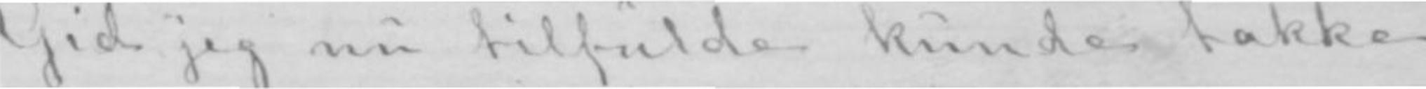Gid jeg nu tilfulde kunde takke
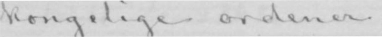kongelige ordener.
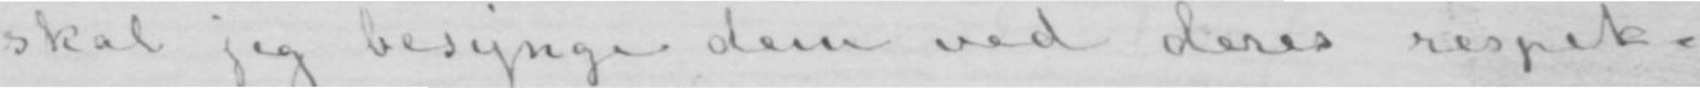skal jeg besynge dem ved deres respek-
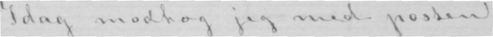Idag modtog jeg med posten
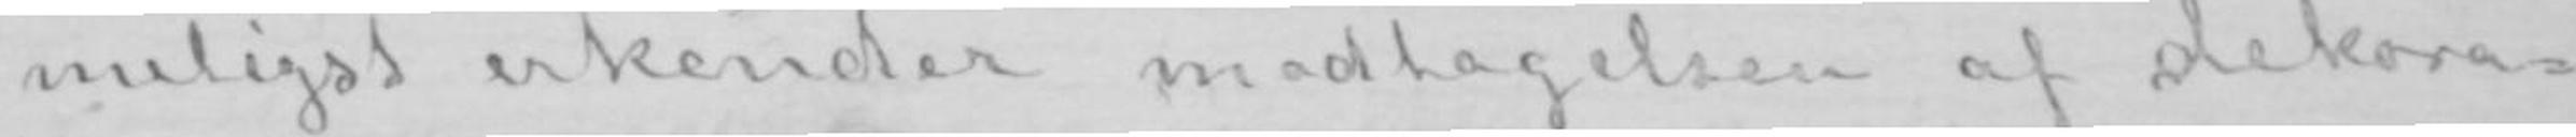meligst erkender modtagelsen af dekora-
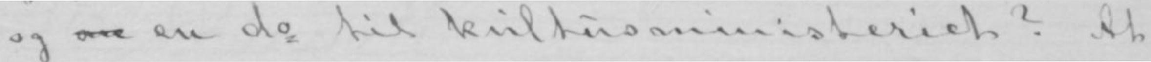og an en do til kultusministeriet? At
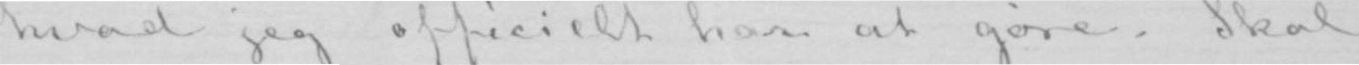hvad jeg officielt har at gøre. Skal
